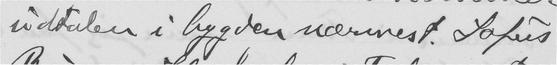udtalen i bygden nærmest. Sofus
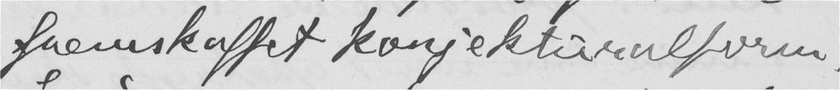fremskaffet konjekturalform.
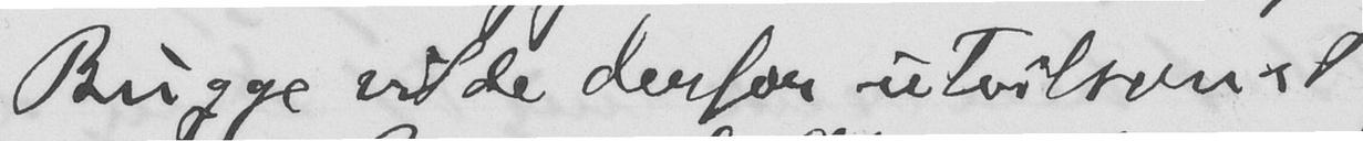Bugge vilde derfor utvilsomt
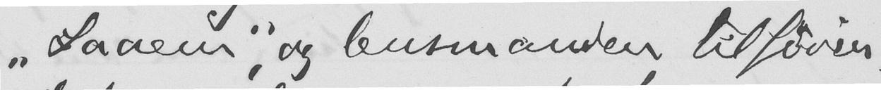"Saaem", og lensmanden tilføier,
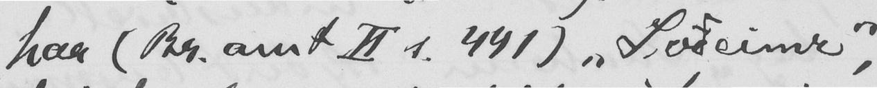har (Br. amt II s. 441) "Soðeimr",
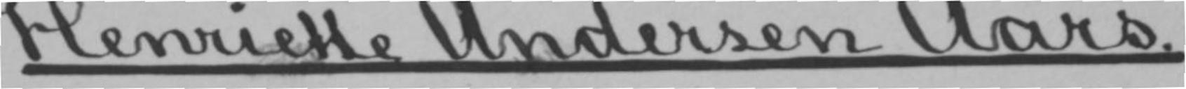Henriette Andersen Aars.
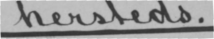hersteds.
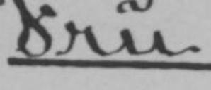Fru
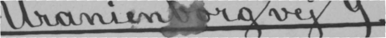Uranienborg vej 9.
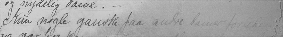Kun nogle ganske faa andre damer foruden
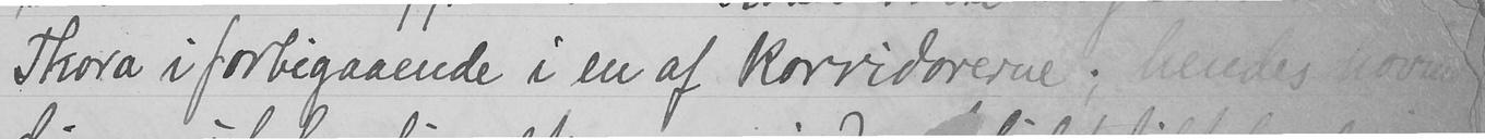Thora i forbigaaende i en af korridorene ; hendes hovmo-
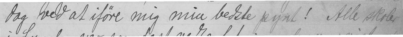dag ved at iføre mig min bedste pynt! Alle skoler
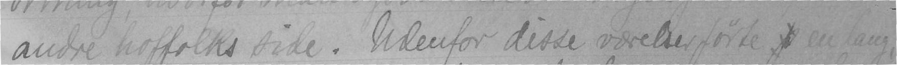andre hoffolks side. Udenfor disse værelser førte jo en lang,
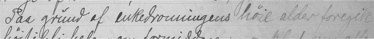Paa grund af enkedronningens høie alder foregik
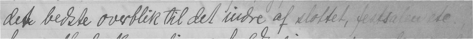det bedste overblik til det indre af slottet, festsalen etc.,
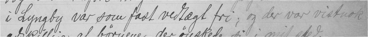i Lyngby var som fast vedtægt fri; og der var vistnok
